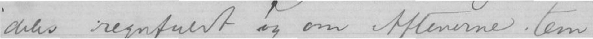deles regnfuldt og om Afterne tem-
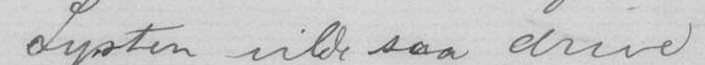Lysten vilde saa drive
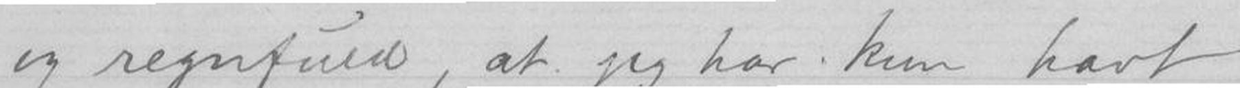regnfuld, at jeg har kun havt
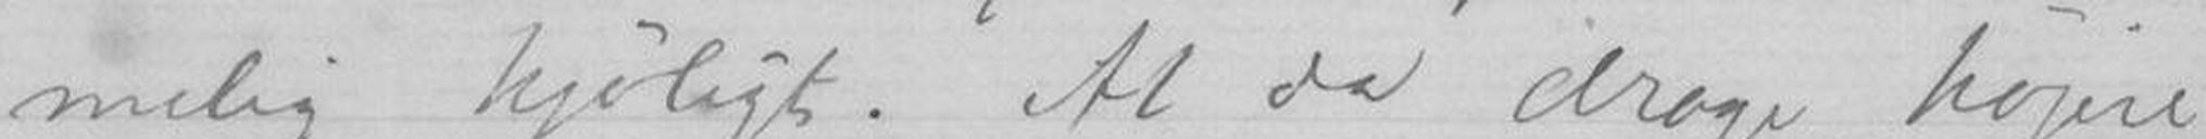melig kjøligt. At da drage højere
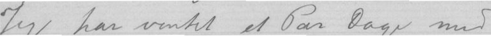Jeg har ventet et Par Dage med
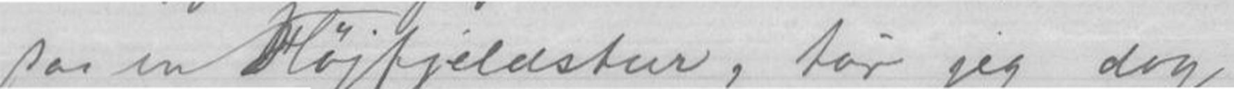paa en Højfjeldstur, tør jeg dog
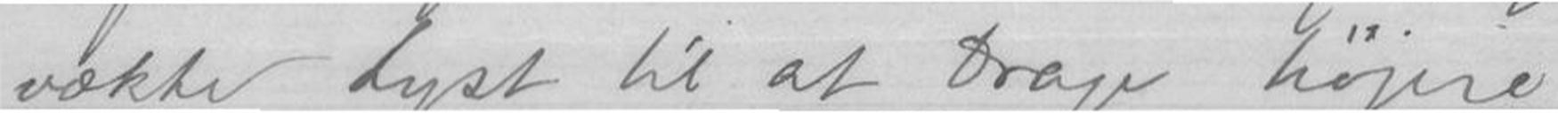vækte Lyst til at Drage Højere
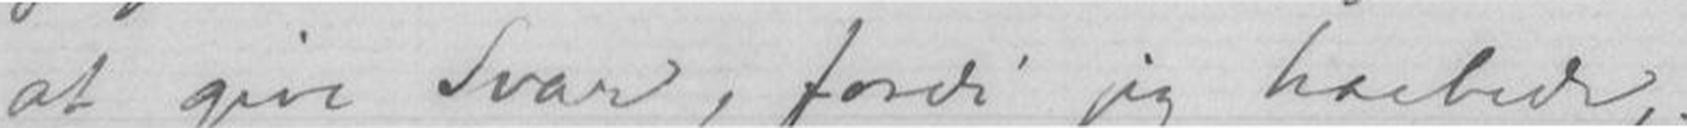at give Svar, fordi jeg haabede,
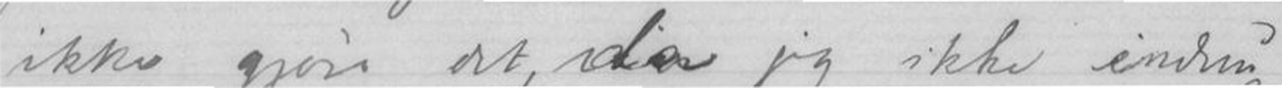ikke gjøre det, da jeg ikke endnu
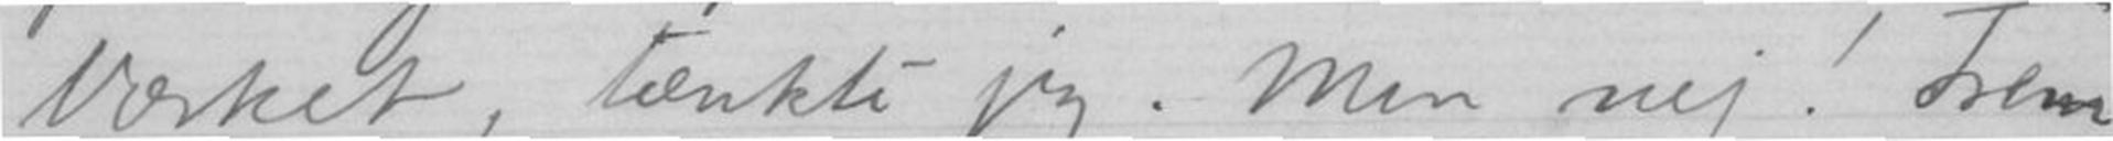Værket, tænkte jeg. Men nej! Frem-
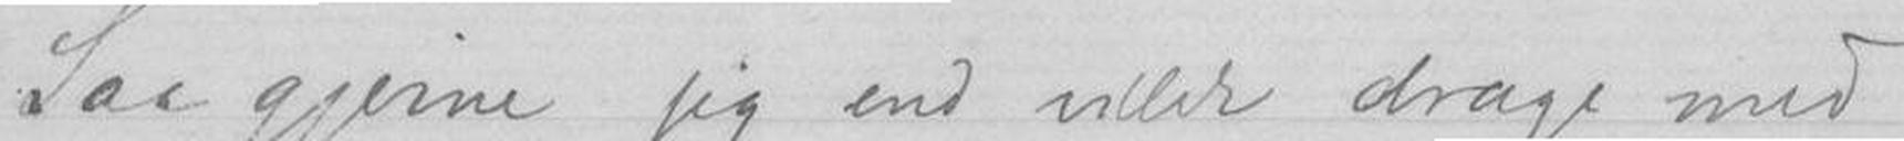Saa gjerne jeg vilde drage med
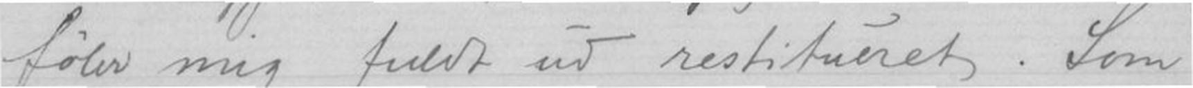føler mig fuldt ud restitueret. Som-
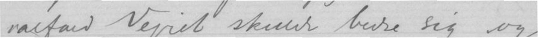ialfald Vejret skulde bedre sig og
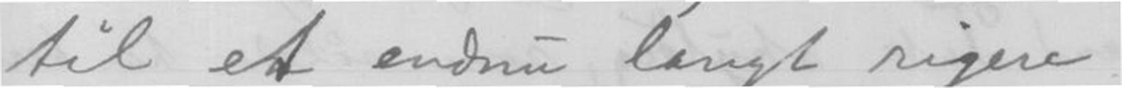til et endnu langt rigere
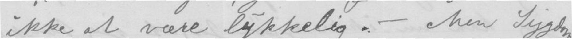ikke at være lykkelig. - Men Sygdom
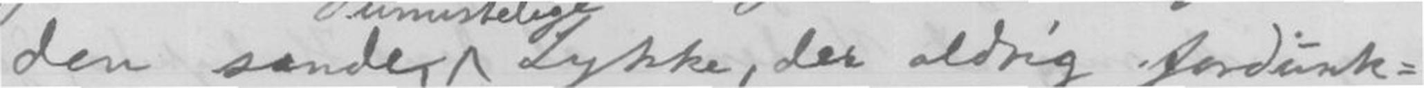den sænder Lykke, der aldrig fordunk-
